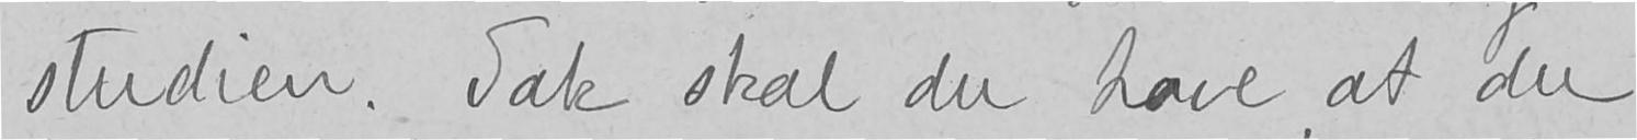studien. Tak skal du have at du
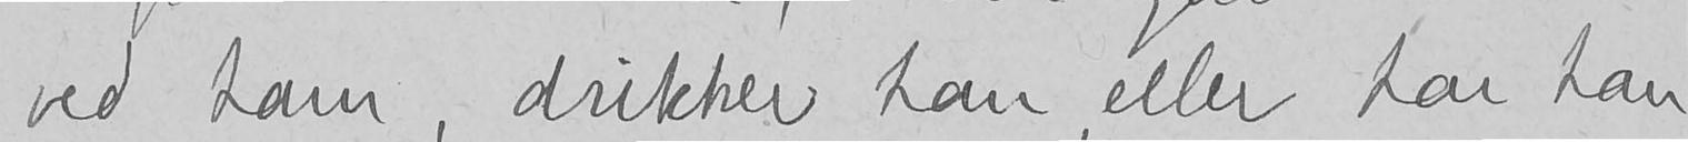ved ham, drikker han, eller har han
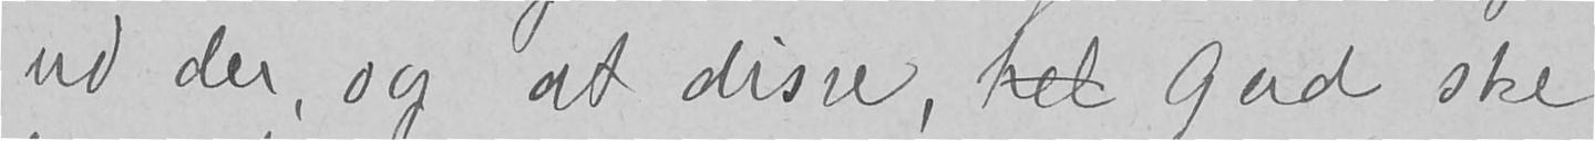ud der, og at disse, hel Gud ske
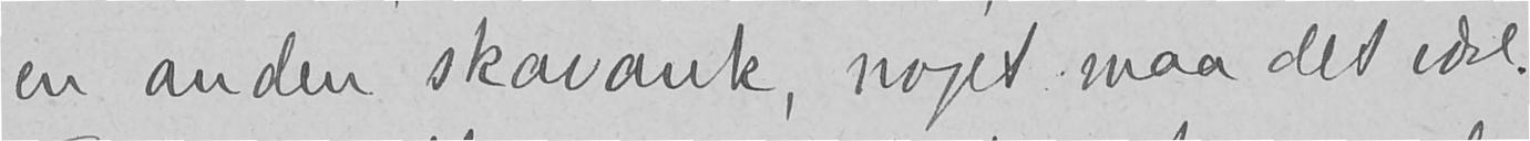en anden skavank, noget maa det være.
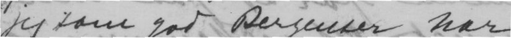jeg som god Bergenser har
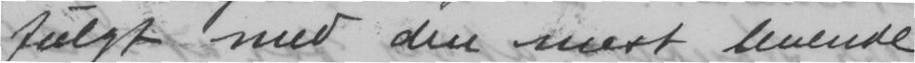fulgt med den svært levende
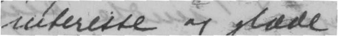interesse og glæde.
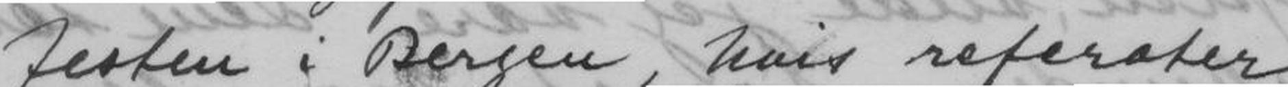festen i Bergen, hvis referater
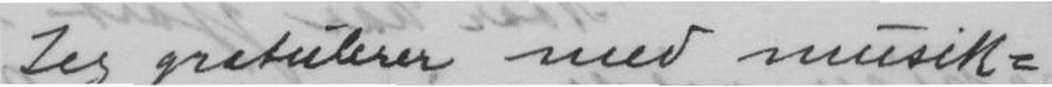Jeg gratulerer med musik-
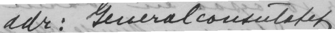adr: Generalconsulatet.
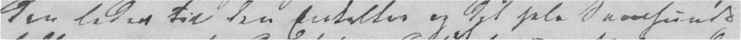den leder til den Enkeltes og det hele Samfunds
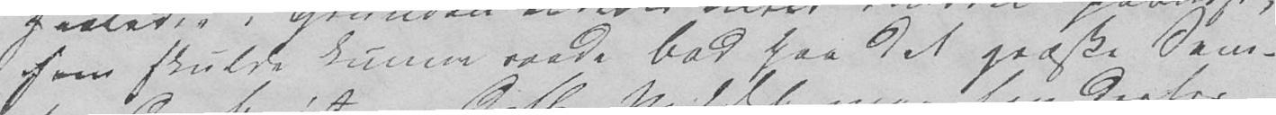som skulde kunne raade Bod paa det græske Sam-
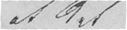at det
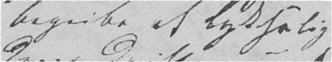begribe at Lyksalig-
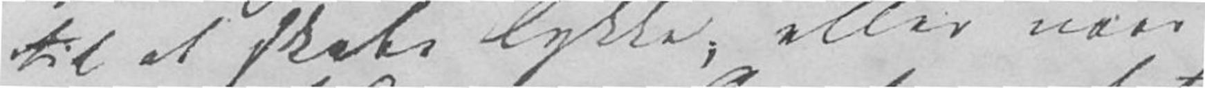til at skabe Lykke; eller naar
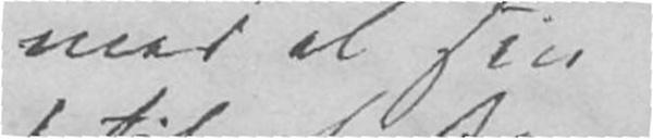med al sin
